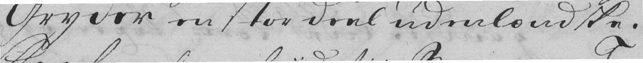Gryder en stor deel udenlændske.
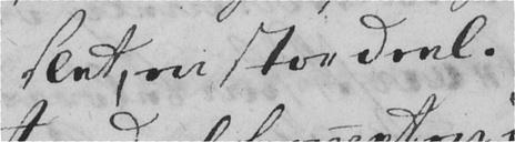slet, en stor deel.
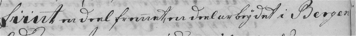fiint en deel fremet, en deel arbeydet i Bergen
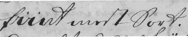fiint mest Sort.
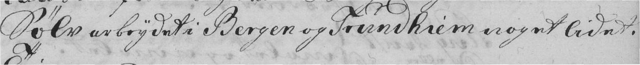Sølv arbeydet i Bergen og Trundhiem noget lidet.
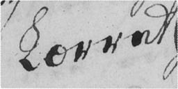Lærret
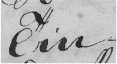Tin
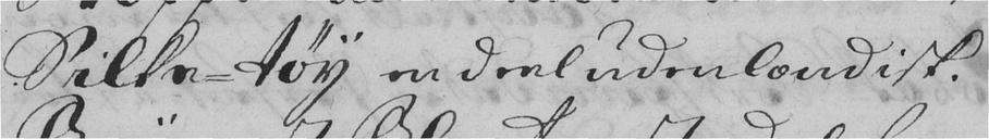Silke-tøy en deel udenlændisk.
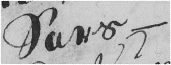Sars
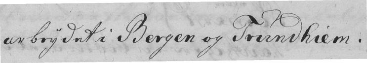arbeydet i Bergen og Trundhiem.
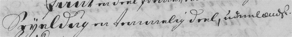Seyeldug en temmelig deel, udenlændsk.
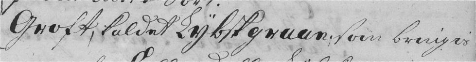Groft, kaldet Lybskgraae, som bringis
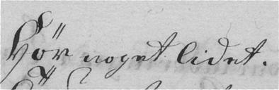Hør noget lidet.
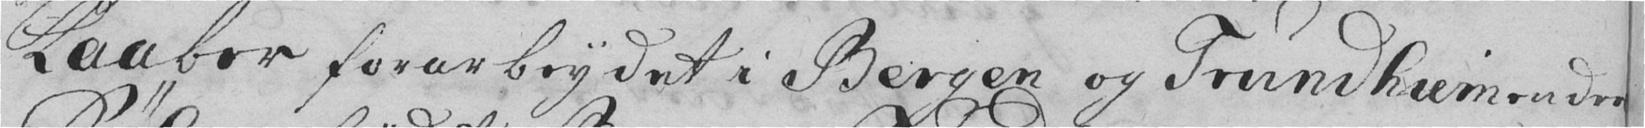Kaaber forarbeydet i Bergen og Trundhiem en deel
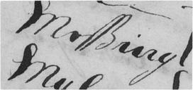Messing
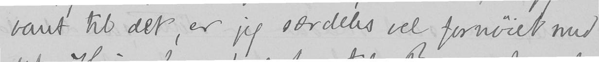vant til det, er jeg særdeles vel fornøiet med
